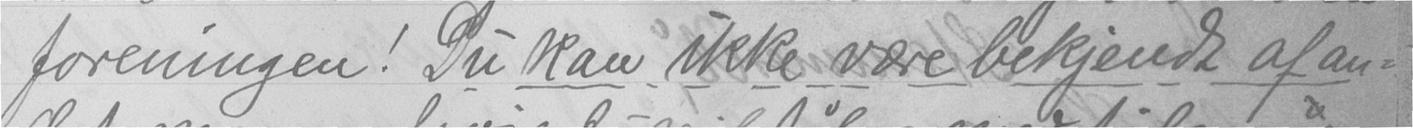foreningen! Du kan ikke være bekjendt af an-
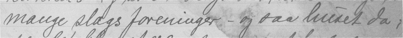mange slags foreninger - og saa huset da;
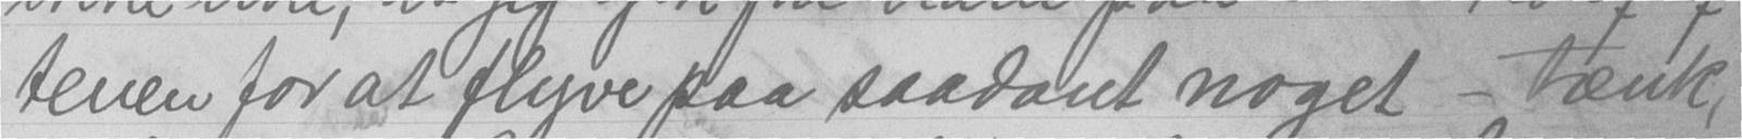tenen for at flyve paa saadant noget - tænk,
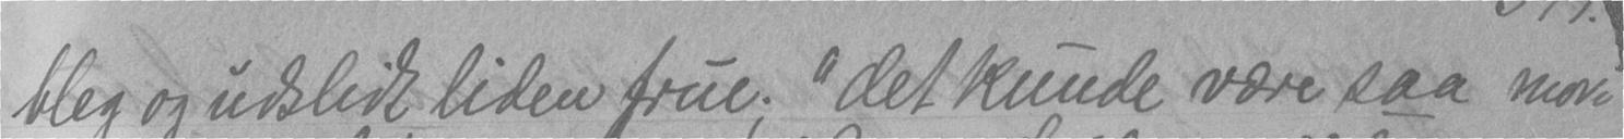bleg og udslidt liden frue; "det kunde være saa mor-
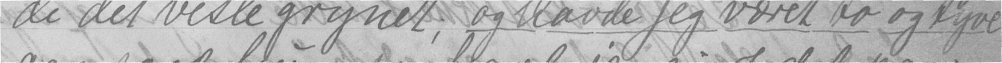de det vesle grynet; og havde jeg været to og tyve
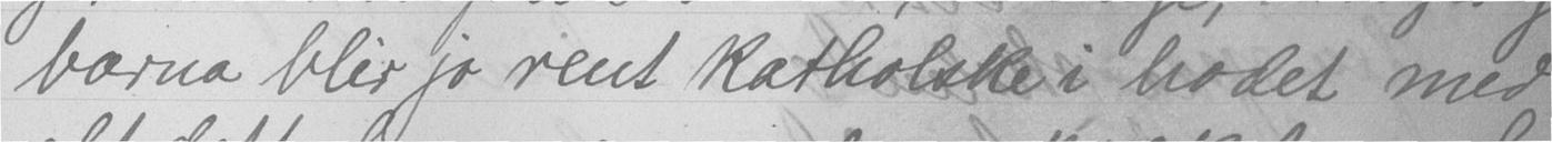barna blir jo rent katholske i hodet med
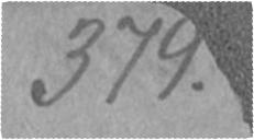374.
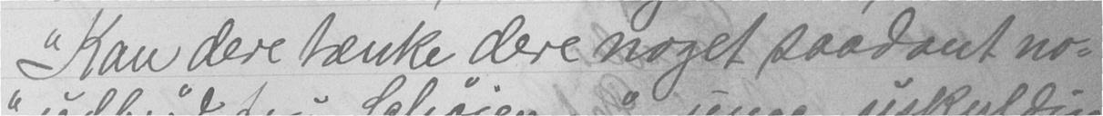"Kan dere tænke dere noget saadant no-
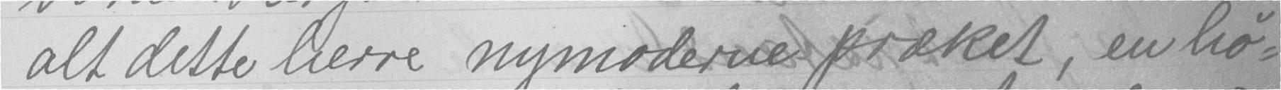alt dette herre nymoderne præket, en hø-
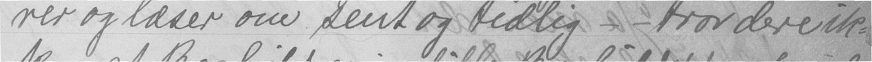rer og læser om sent og tidlig - - tror dere ik-
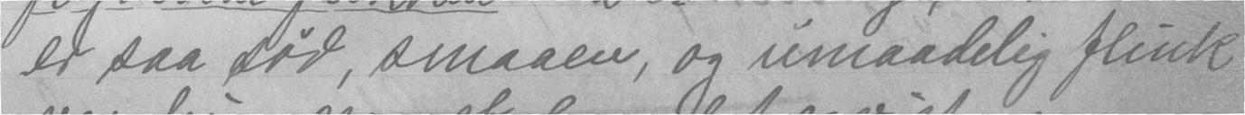er saa sød, smaaen, og umaadelig flink
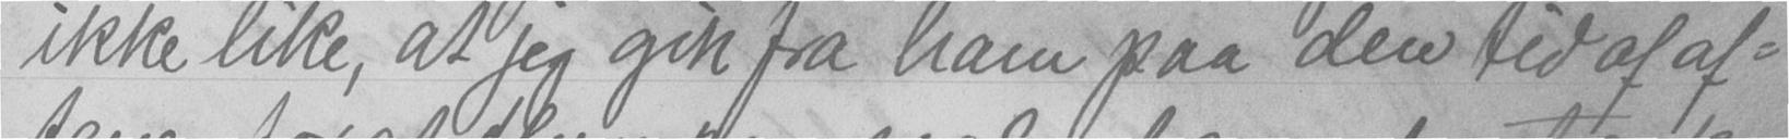ikke like, at jeg gik fra ham paa den tid af af-
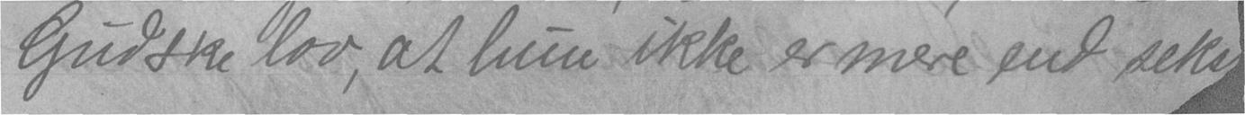Gudske lov, at hun ikke er mere end seks
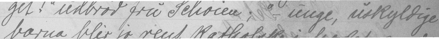get!" udbrød fru Schøien; " - unge, uskyldige
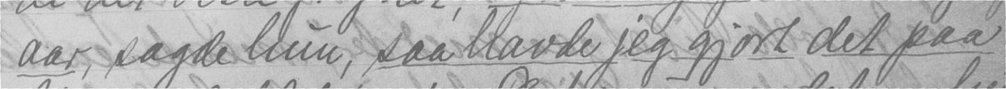aar, sagde hun, saa havde jeg gjort det paa
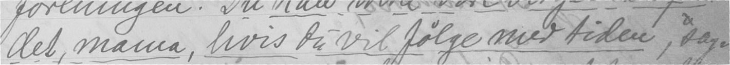det, mama, hvis du vil følge med tiden, sag-
